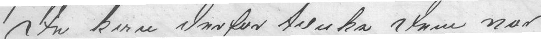De kan derfor tænke Dem vor
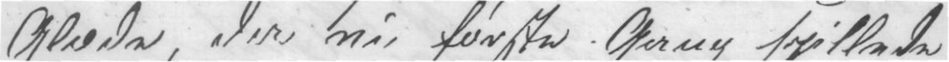Glæde, der vi første Gang spillede
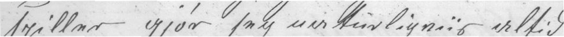spiller gjør jeg naturligvis altid
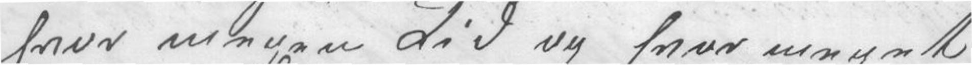hvor megen Tid og hvor meget
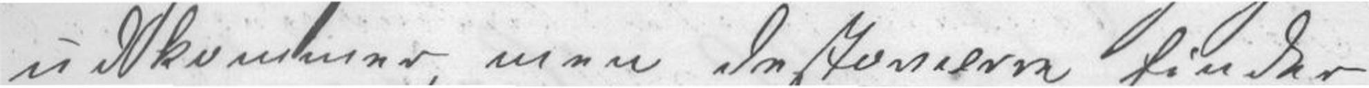udkommer, men destoværre finder
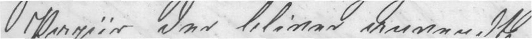Papiir der bliver anvendt.
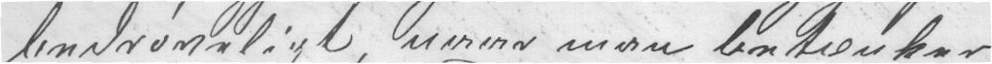bedrømeligt, naar man betænker
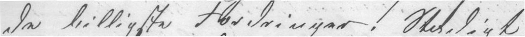de billigste Fordringer. Stadigt
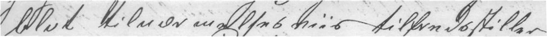blot tilnærmelsesviis tilfredsstiller
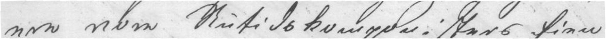ere vore Nutidskomponisters Eien-
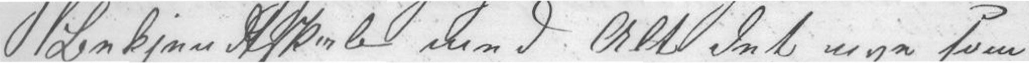Bekjendtskab med Alt det nye som
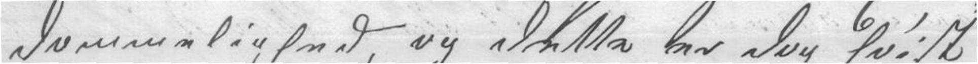dommelighed og dette er dog høit
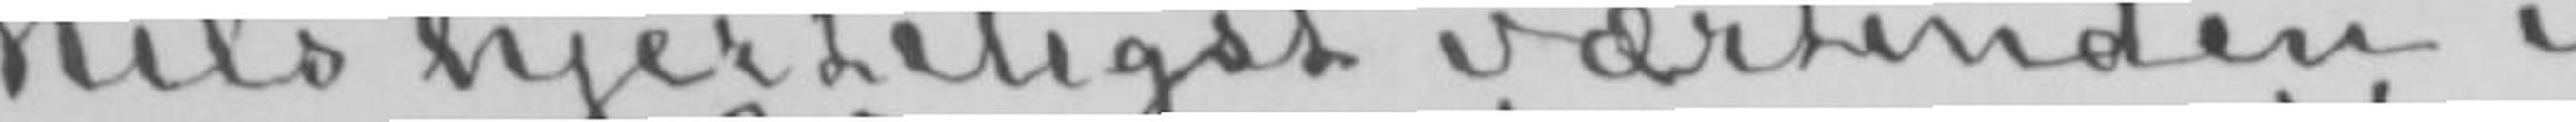Hils hjerteligst værtinden i
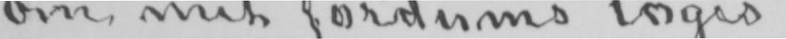om mit fordums logis.
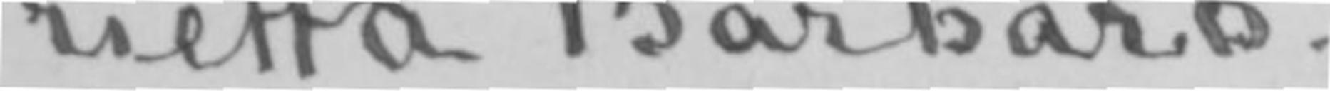rietta Barbaro.
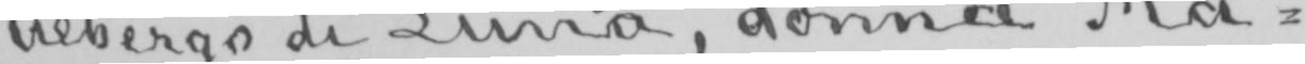Albergo di Luna, donna Ma-
